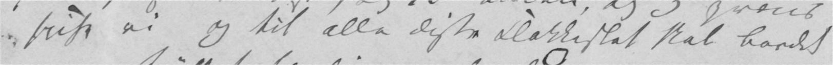spise vi og til alle disse Klokkeslet skal Bordet
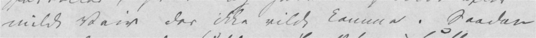mildt Veir der ikke vilde komme. Saadan
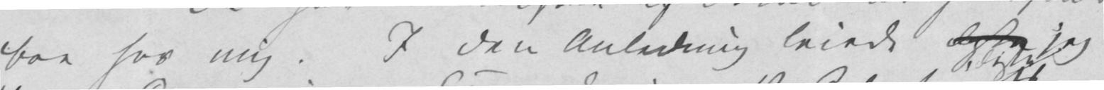boe hos mig. I den Anledning leiede disse jeg
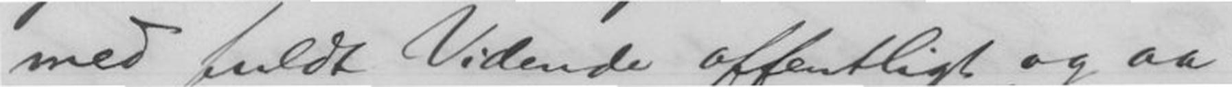med fuldt Vidende offentligt og aa-
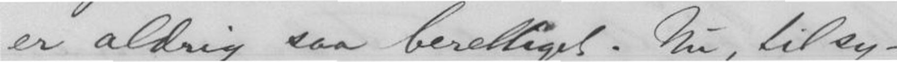er aldrig saa berettiget. Nu, til sy-
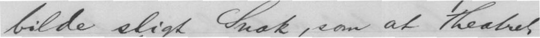bilde sligt Snak, som at Theatret
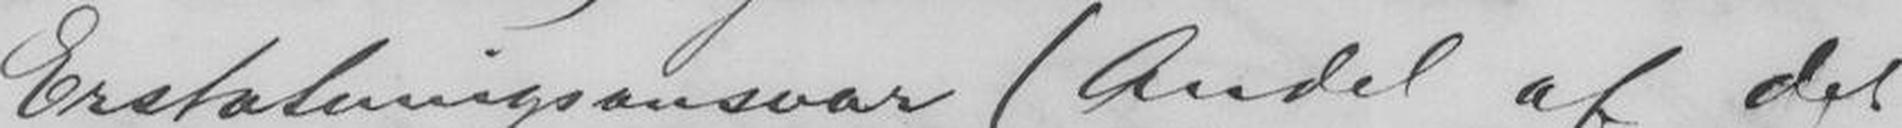Erstatningsansvar (Andel af det
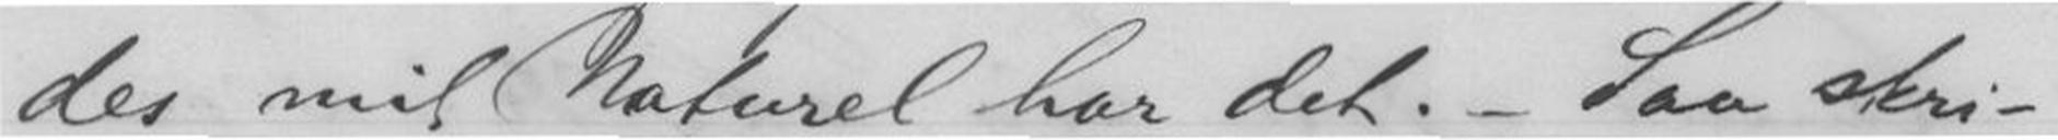des mit Naturel har det. - Saa skri-
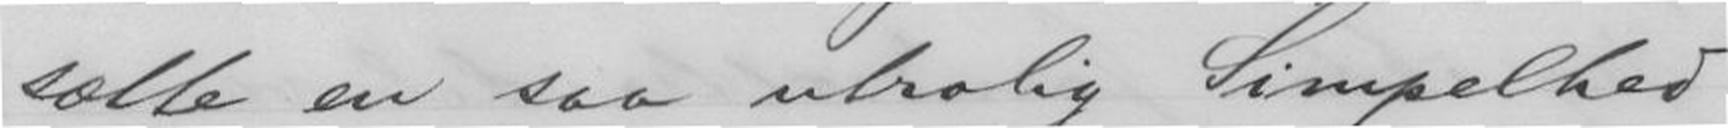sætte en saa utrolig Simpelhed
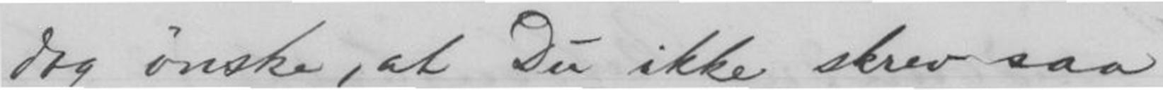dog ønske, at Du ikke skrev saa
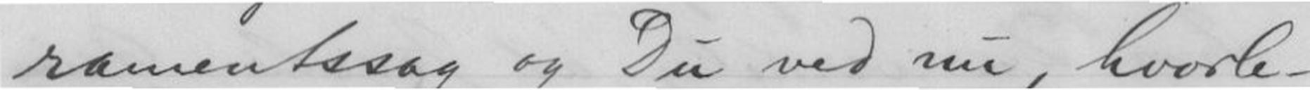ramentssag og Du ved nu, hvorle-
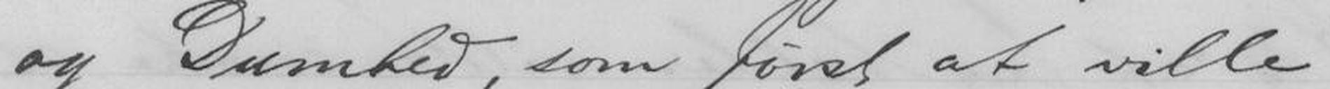og Dumhed, som først at ville
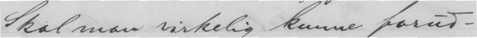Skal man virkelig kunne forud-
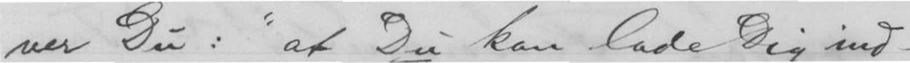ver Du: "at Du kan lade Dig ind-
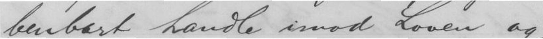benbart handle imod Loven og
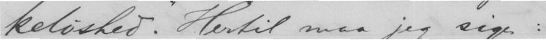keløshed." Hertil maa jeg sige:
